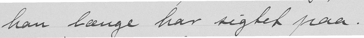han længe har sigtet paa.
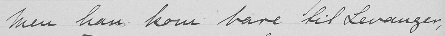Men han kom bare til Levanger,
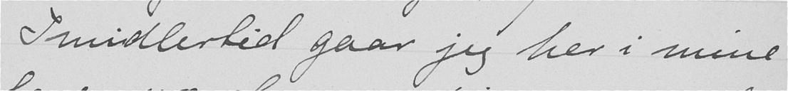Imidlertid gaar jeg her i mine
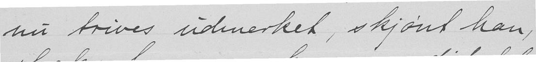nu trives udmerket, skjønt han,
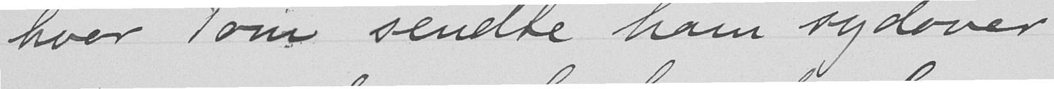hvor Tom sendte ham sydover
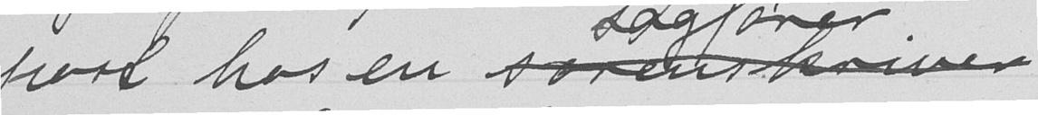post hos en sorenskriver
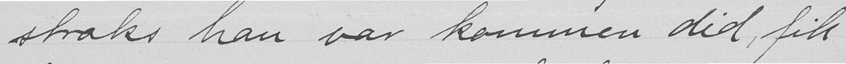straks han var kommen did, fik
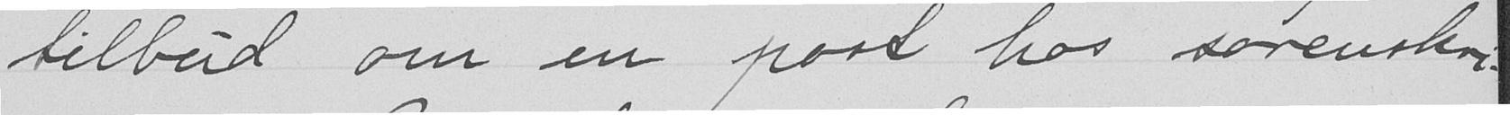tilbud om en post hos sorenskri-
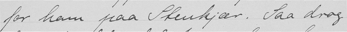for ham paa Stenkjær. Saa drog
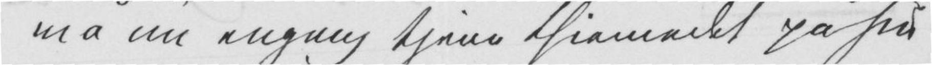må nu engang tjene Øiemedet på sin
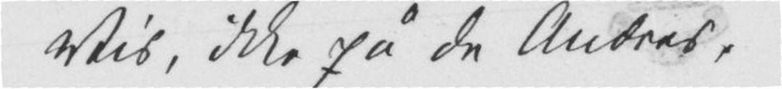Vis, ikke på de Andres.
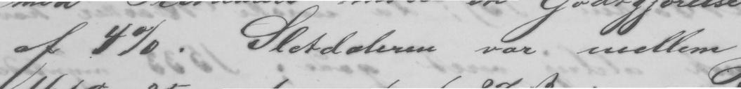af 4%. Slatdaleren var mellem
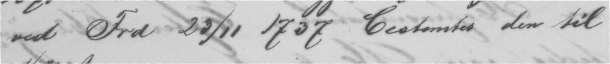ved Frd 23/11 1737 bestemtes den til
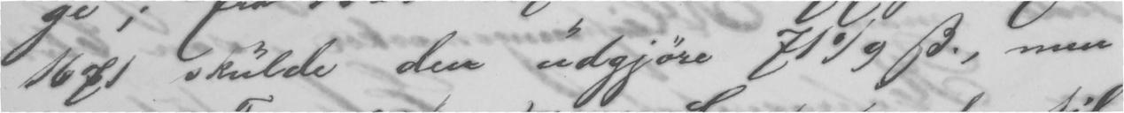1671 skulde den udgjøre 71% ẞ., men
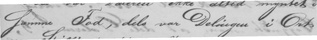Samme Fod, dels var Delingen i Ort
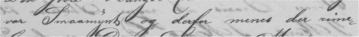var Smaamynt og derfor menes der rime-
